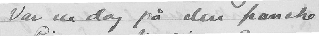Var en dag på den franske
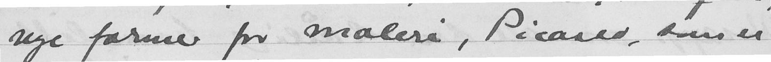nye former for maleri, Picasso, som er
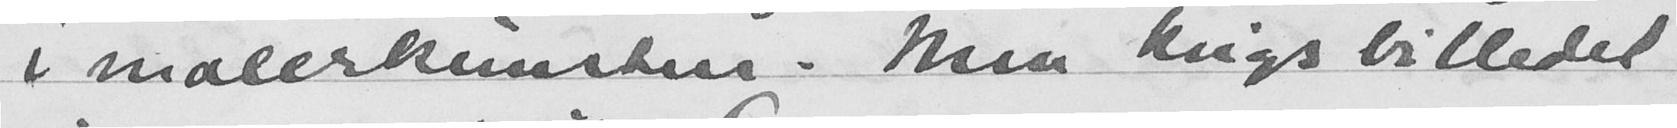i malerkunsten. Men krigsbilledet
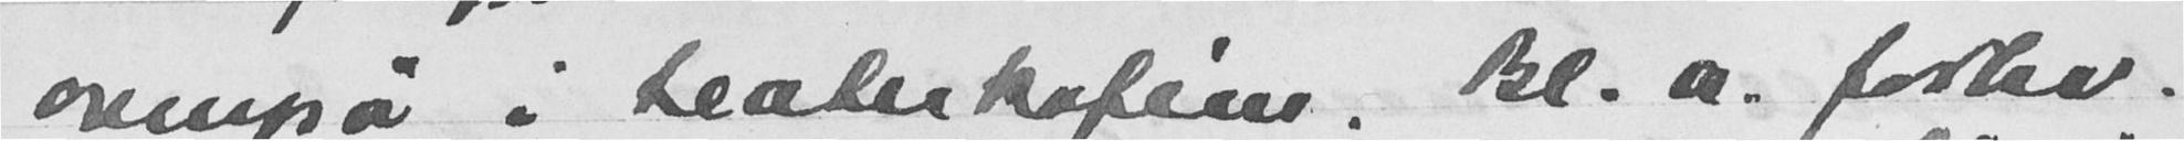ovenpå i teaterkafeen. Bl. a. forblev.
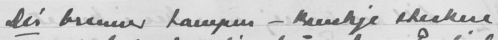Der brenner tampen - kanskje sterkere
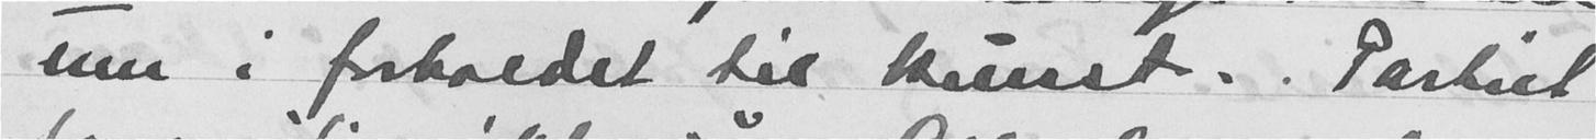enn i forholdet til kunst. Partiet
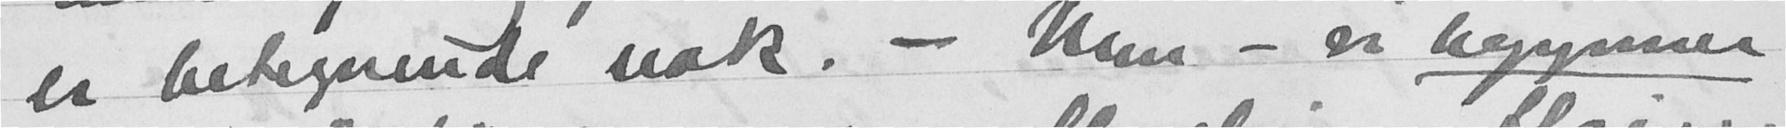er betegnende nok. - Men - vi begynner
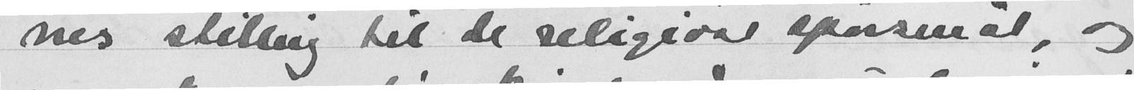nes stilling til de religiøse spørsmål, og
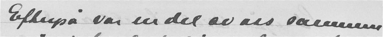Efterpå var en del av oss sammen
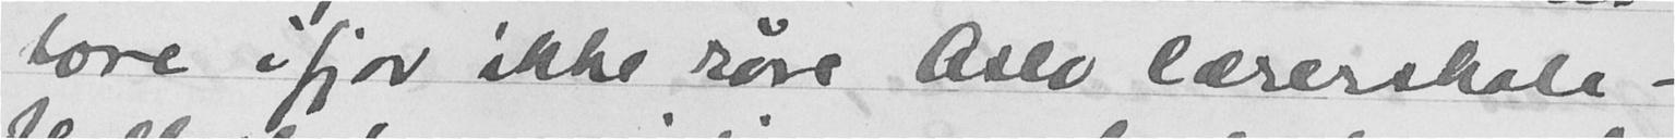løre ifjor ikke røre Oslo lærerskole -
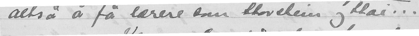altså å få lærere som Storstein og Stai...
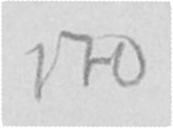170
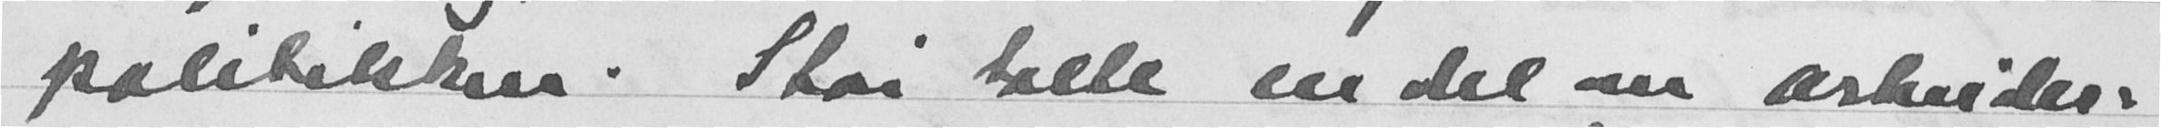politikken. Stai talte en del om arbeider-
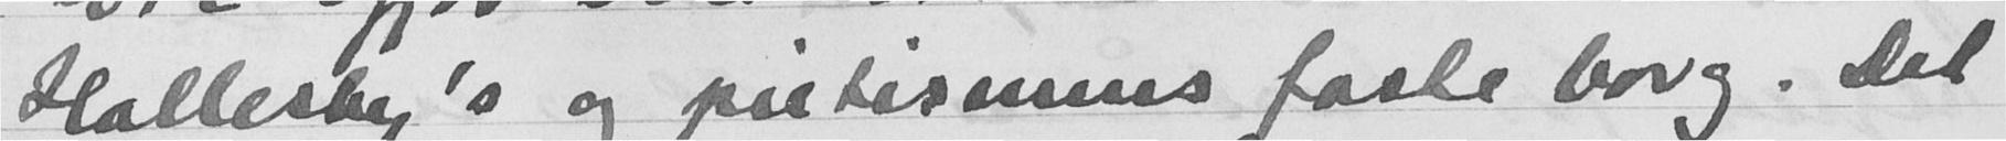Hallesby's og pietismens faste borg. Det
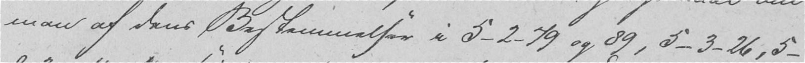man af dens Bestemmelser i 5-2-79 og 89, 5-3-26, 5-
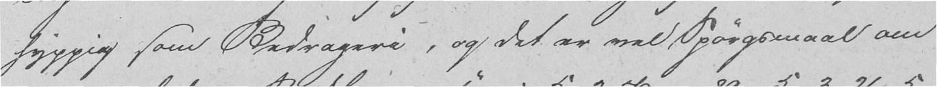hyppig som Bedrageri, og det er vel Spørgsmaal om
